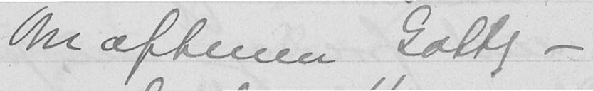Om aftenen Gottf -
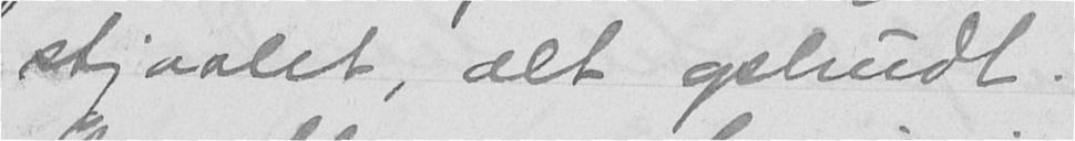stjaalet, alt opbrudt.
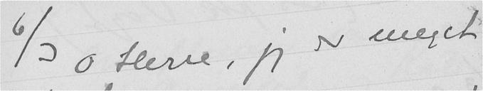6/3 O Herre, jeg er meget
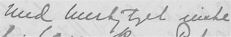Med hurtigtoget reiste
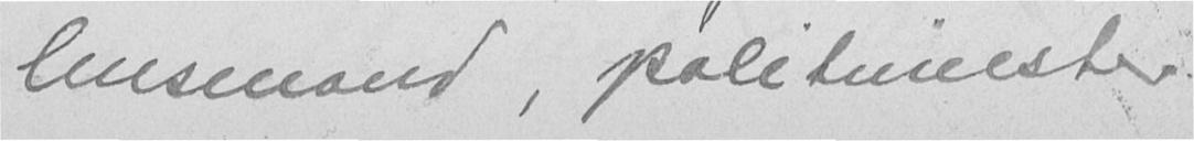lensmand, politimester.
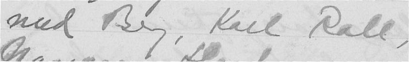med Berg, Karl Roll,
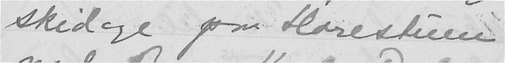skidage paa Harestuen
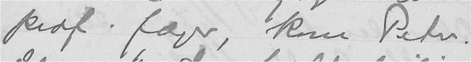prof. Jæger, kom Peter.
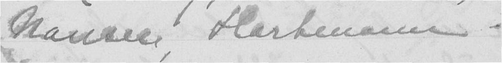Nansen, Hartmann
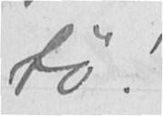tø!
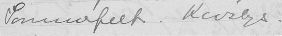Sommerfelt. Krosbys.
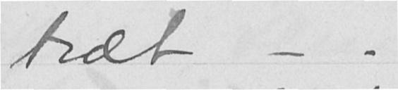træt - .
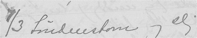7/3 Søndenstorm og slig
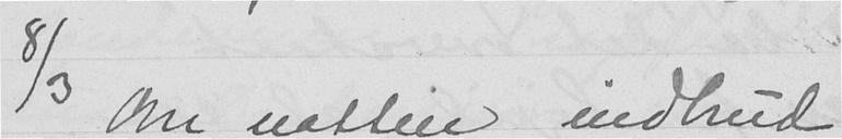8/3 Om natten indbrud
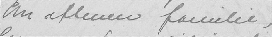Om aftenen familie,
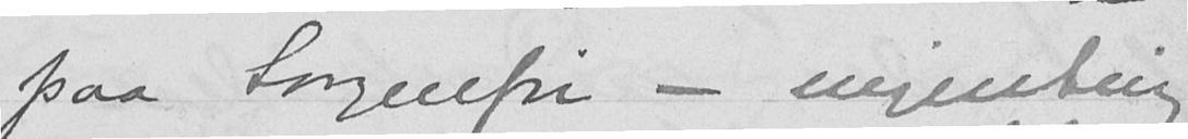paa Sorgenfri - ingenting
